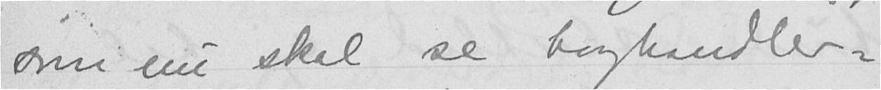som nu skal se boghandler-
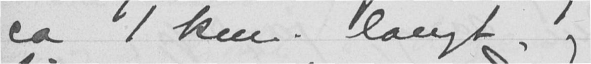ca 1 km. langt, og
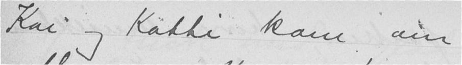Kai og Katti kom om
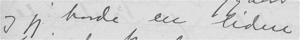og jeg havde en liden
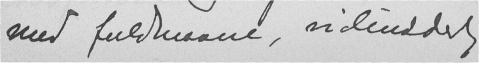med fuldmaane, vidunderlig
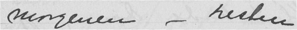morgenen - resten
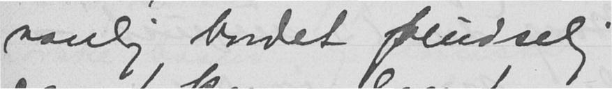vanlig bordet pludselig
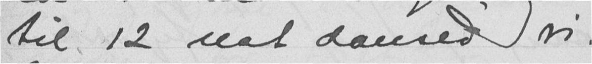til 12 nat dansed vi.
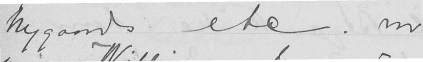Nygaards etc. var
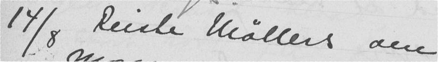14/8 Reiste Møllers om
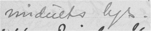vinduets lys.
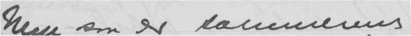Men saa er sommerens
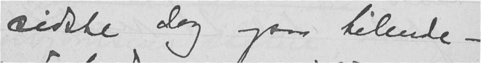sidste dag ogsaa tilende -
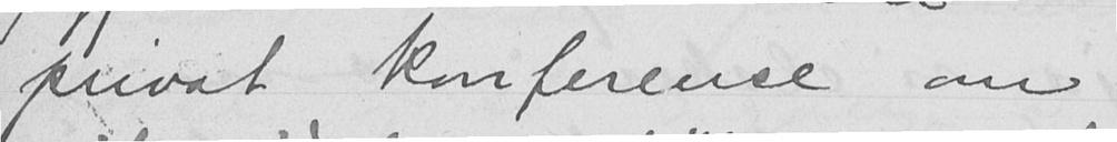privat konferense om
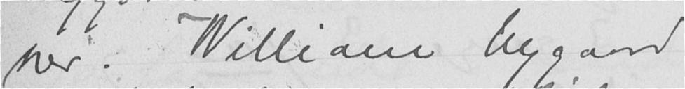her. William Nygaard
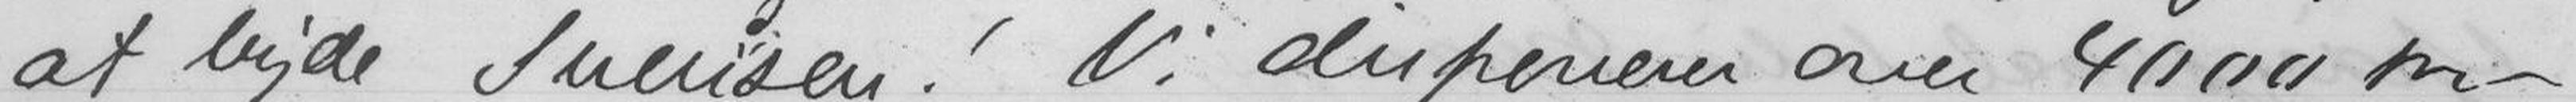at byde Svendsen! Vi disponerer over 4000 kr
